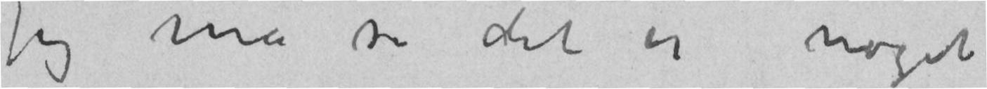Jeg må si det er noget
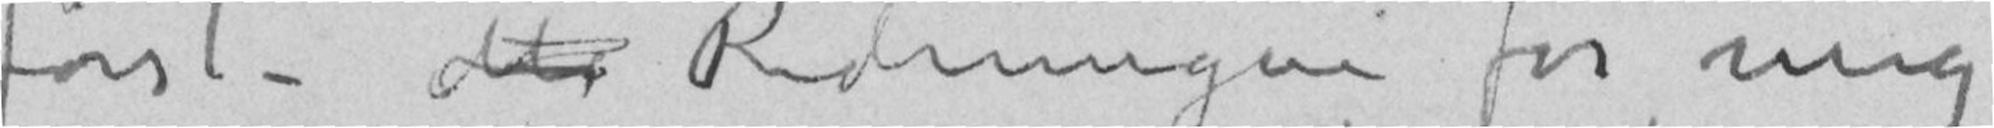først – Redningen for mig
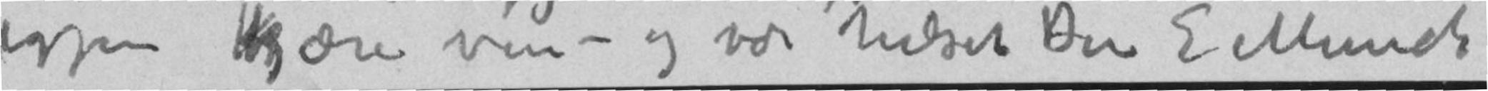igjen Kjære Ven – og vær hilset Din E Munch
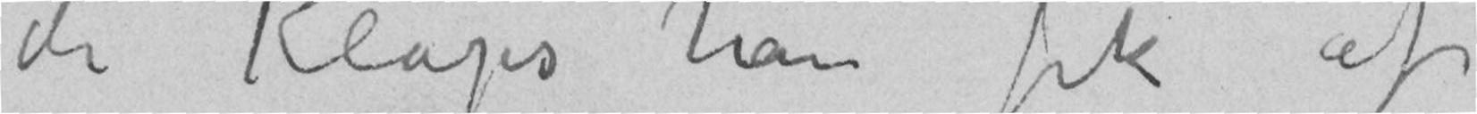de Klaps han fik af
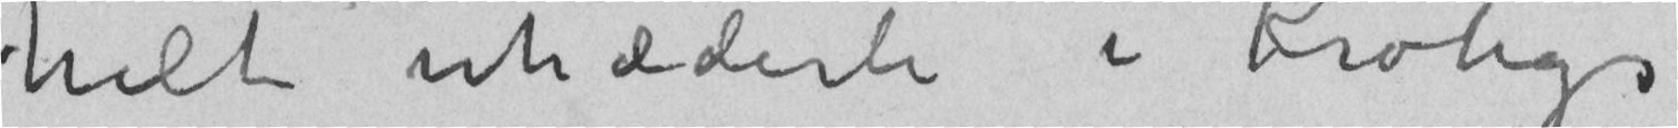helt uhæderli i Krohgs
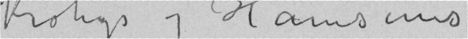Krohgs og Hamsuns
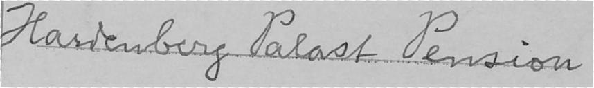Hardenberg Palast Pension
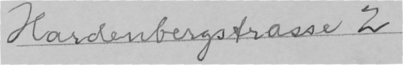Hardenbergstrasse 2
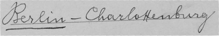Berlin - Charlottenburg
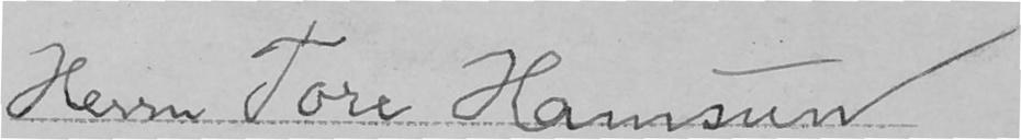Herr Tore Hamsun
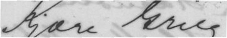Kjære Grieg
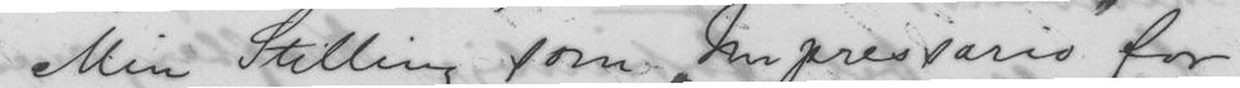Min Stilling som "Impressario" for
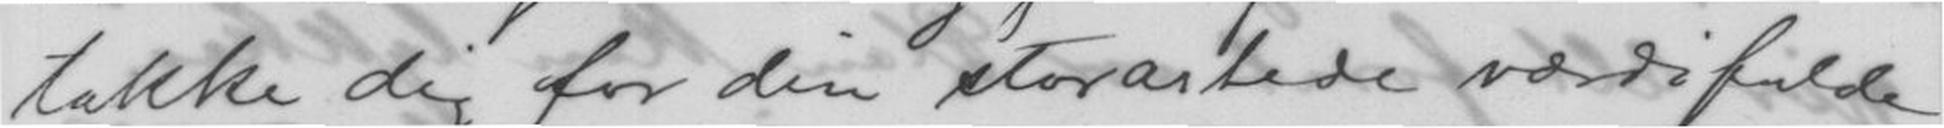takke dig for din storartede værdifulde
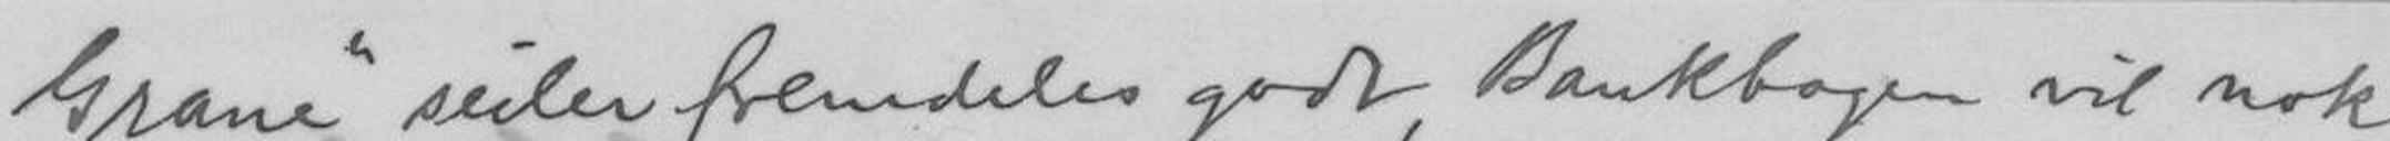Grane seiler fremdeles godt, Bankbogen vil nok
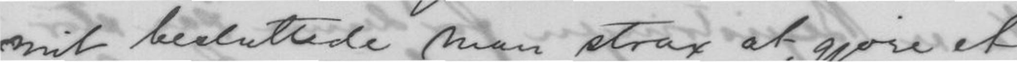mit besluttede man strax at gjøre et
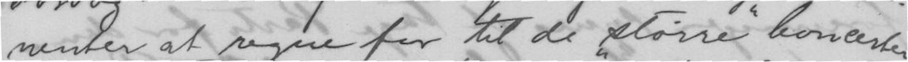nenter at regne for til de større Concerter
